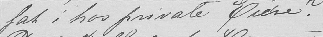fat i hos private Eiere?
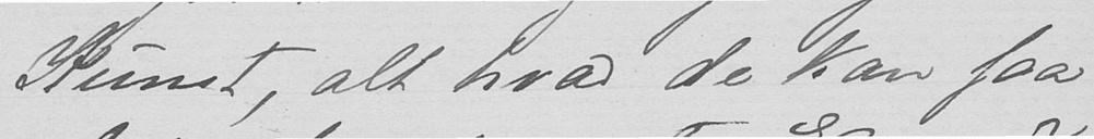Kunst, alt hvad de kan faa
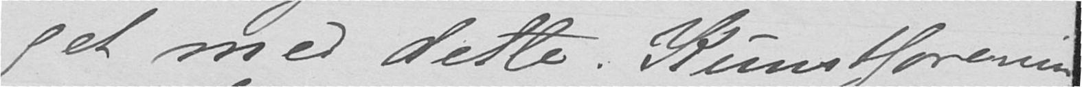get med dette. Kunstforenin-
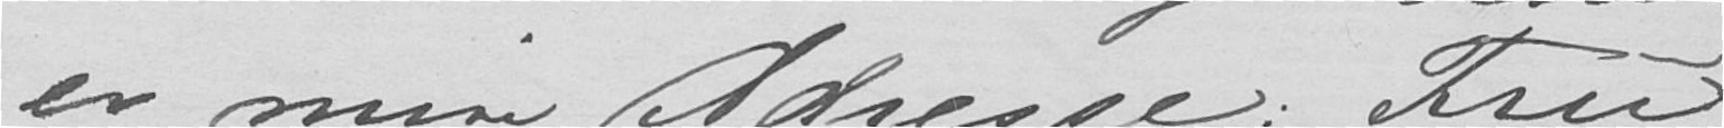er min Adresse: Fru
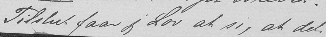Tilslut faar jeg Lov at si, at det
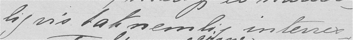ligvis taknemlig interres-
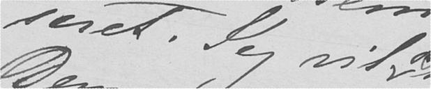seret. Jeg vil
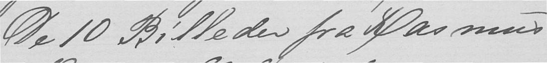De 10 Billeder fra Rasmus
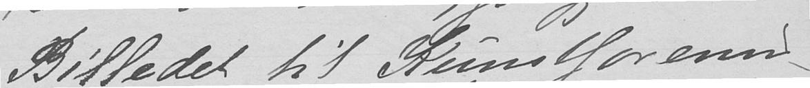Billedet til Kunstforenin-
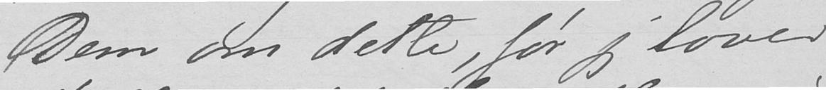Dem om dette, før jeg lover
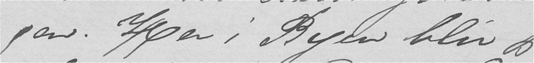gen. Her i Byen blir jeg
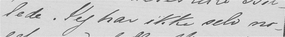lede. Jeg har ikke selv no-
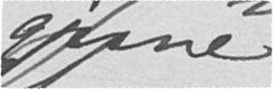gjerne
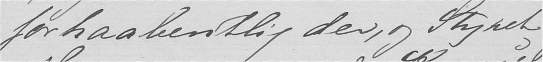forhaabentlig der, og Styret
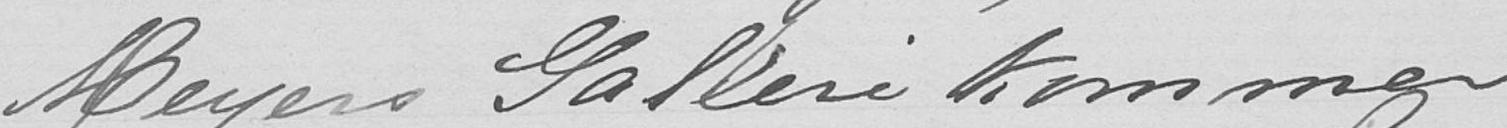Meyers Galleri kommer
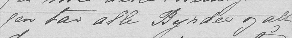gen tar alle Byrder og alt
